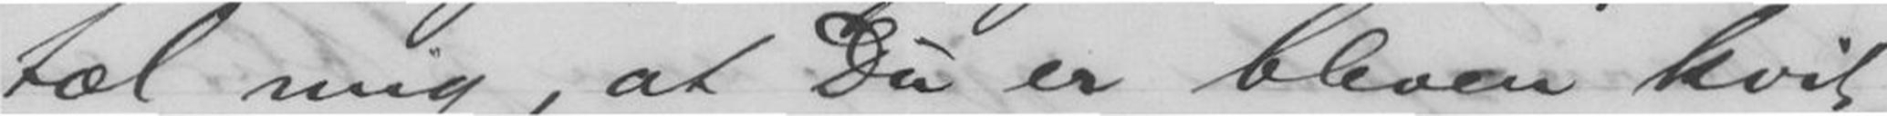tæl mig, at Du er bleven kvit
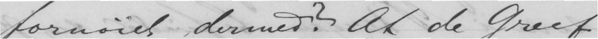fornøiet dermed? At de Greef
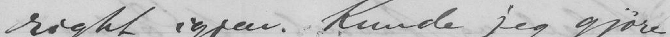right igjen. Kunde jeg gjøre
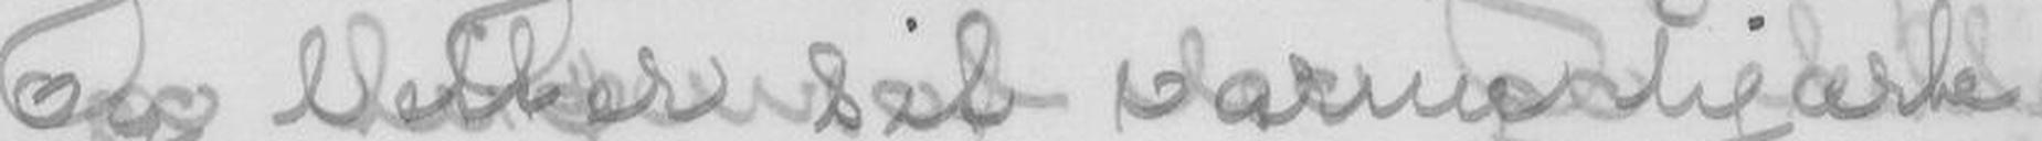og letter sit varme hjærte
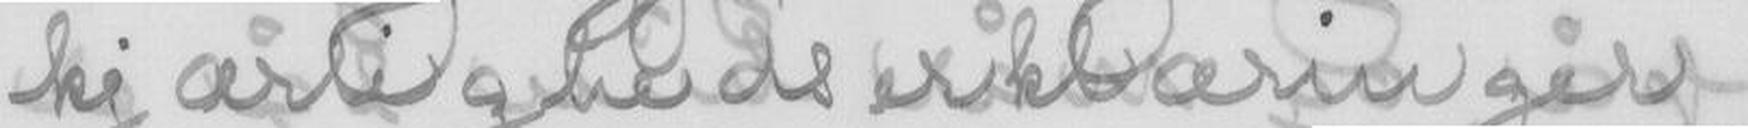kjærlighedserklæringer
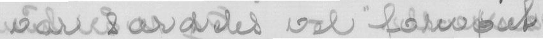var særdeles vel fornøiet
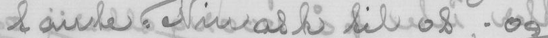tante Ninask til os, - og
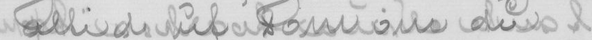altid ut som om du
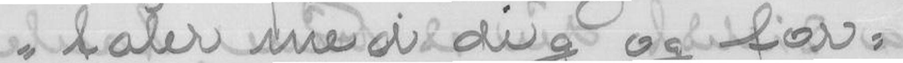taler med dig og for-
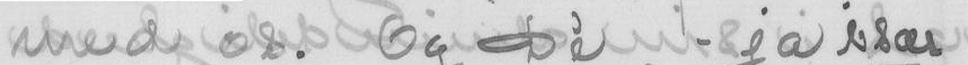med os. Og Dé, - ja især
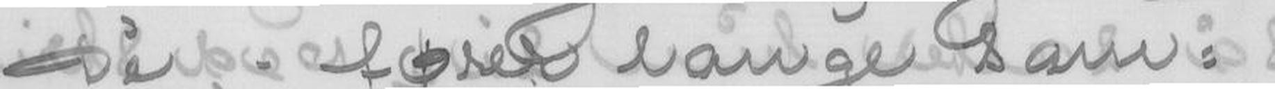Dé - fører lange sam-
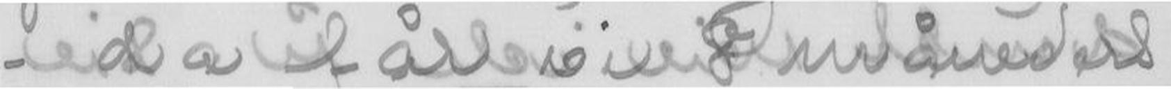_ da får vi 8 måneders
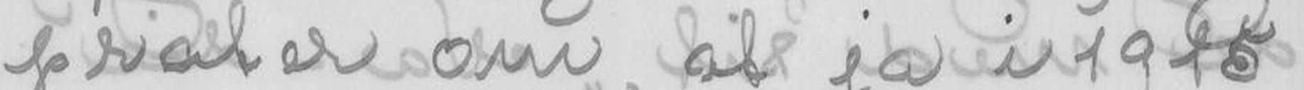prater om, at ja i 1915
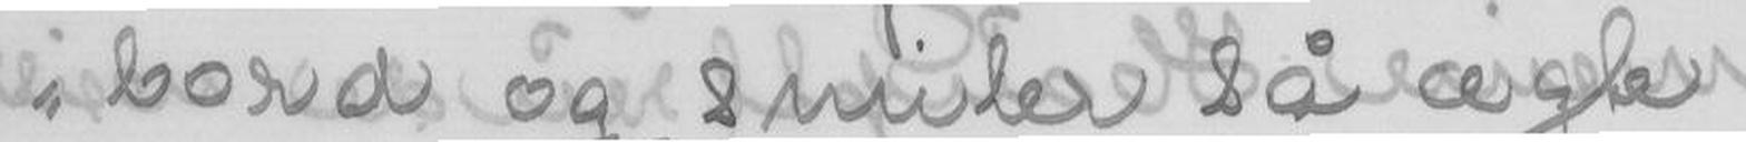bord og smiler så ægte
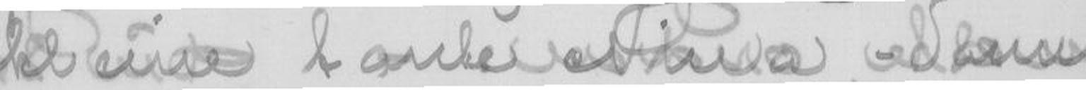kleine tante Nina, - dann
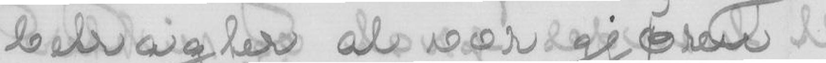betragter al vor gjøren
